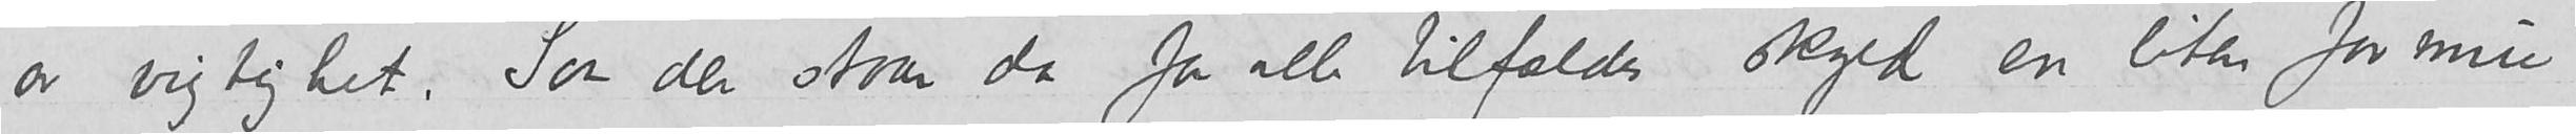av vigtighet. Saa der staar da for alle tilfældes skyld en liten formue
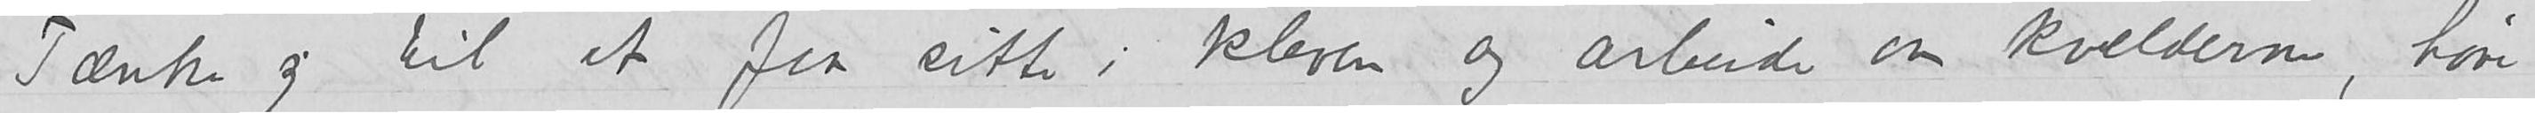Tænke sig til at faa sitte i kleven og arbeide om kvelderne, høre
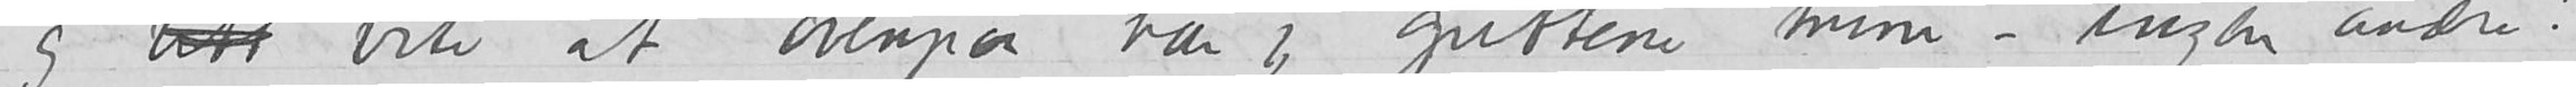og litt vite at ovenpaa har jeg guttene mine – ingen andre!
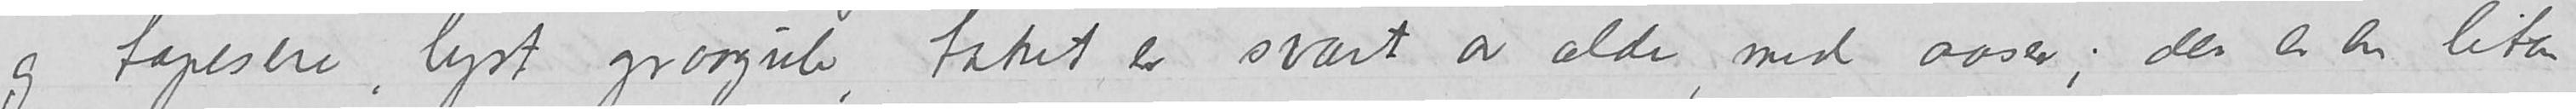og tapesere, lyst graagule, taket er svart av alder, med aaser, der er en liten
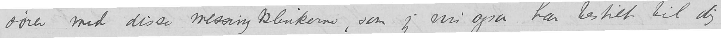dører med disse messingklinkerne, som jeg nu ogsaa har bestilt til dig
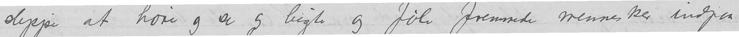slippe at høre og se og lugte og føle fremmede mennesker indpaa
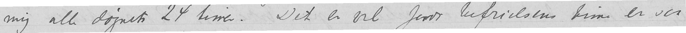mig alle døgnets 24 timer. Det er vel fordi befrielsens time er saa
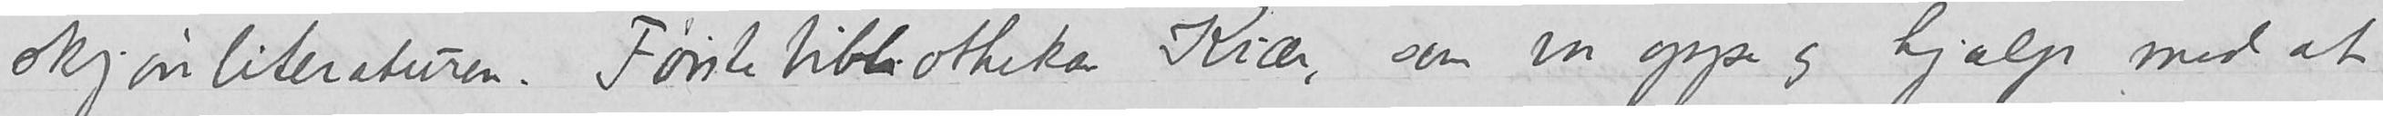skjønliteraturen. Førstebibliothekar Kiær, som var oppe og hjalp med at
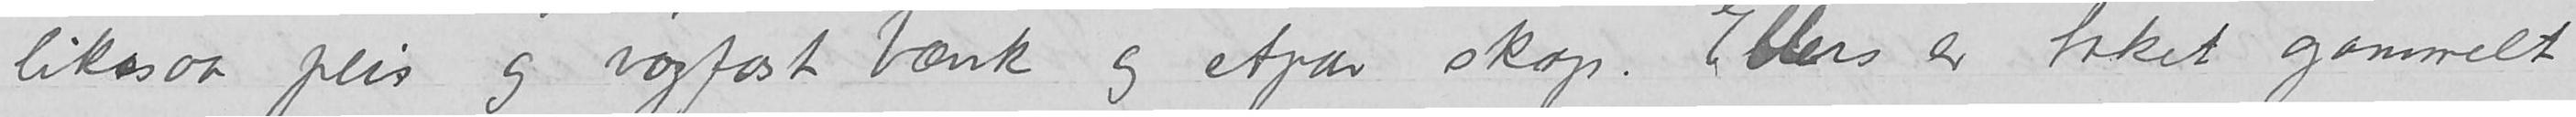likesaa peis og vægfast bænk og etpar skap. Ellers er taket gammelt
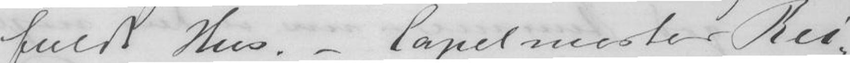fuldt Hus. - Capelmester Rei-
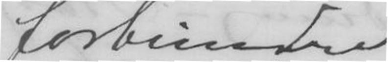forbundne
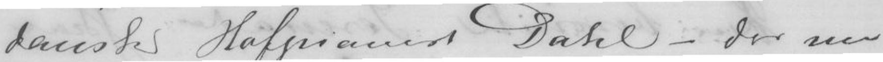dansk Hofpianist Dahl - der nu
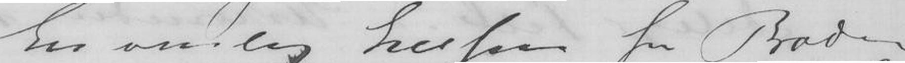En venlig hilsen fra Broder
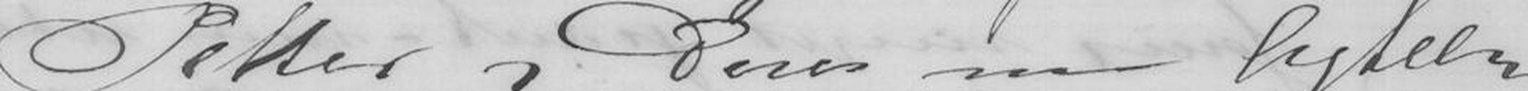Petter og Deres nu Agtelse
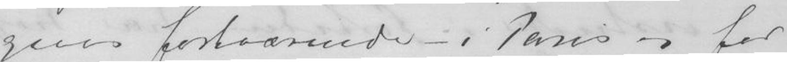gives fortaarende - i Paris og for
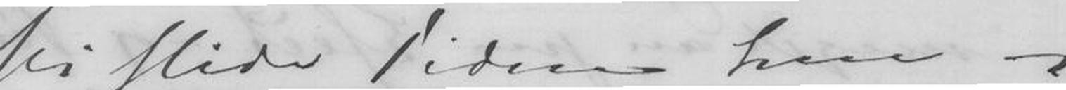Vi slider Tiden hen og
